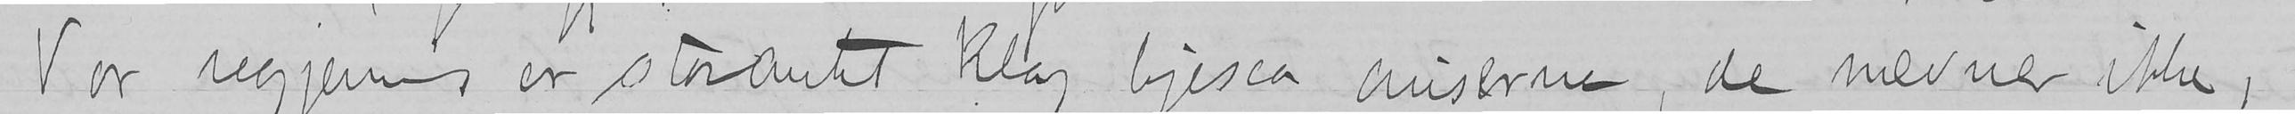Vor regjering er storartet klog ligesaa aviserne, de nevner ikke,
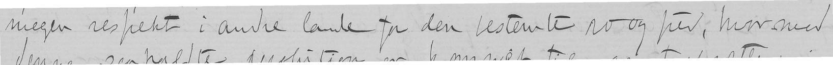megen respekt i andre lande for den bestemte ro og fred, hvormed
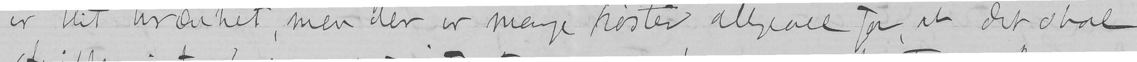er blit krænket, men der er mange røster alligevel for at det skal
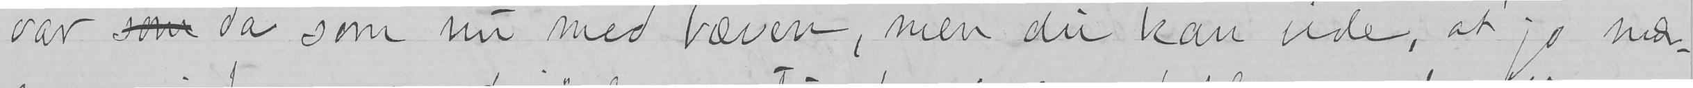var som da som nu med bæven, men du kan vide, at jeg nær-
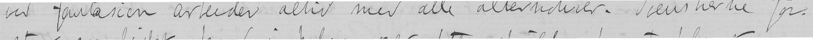ved fantasien arbeider altid med alle alternativer. Svenskerne for-
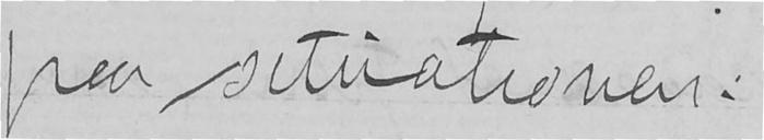paa situationen.
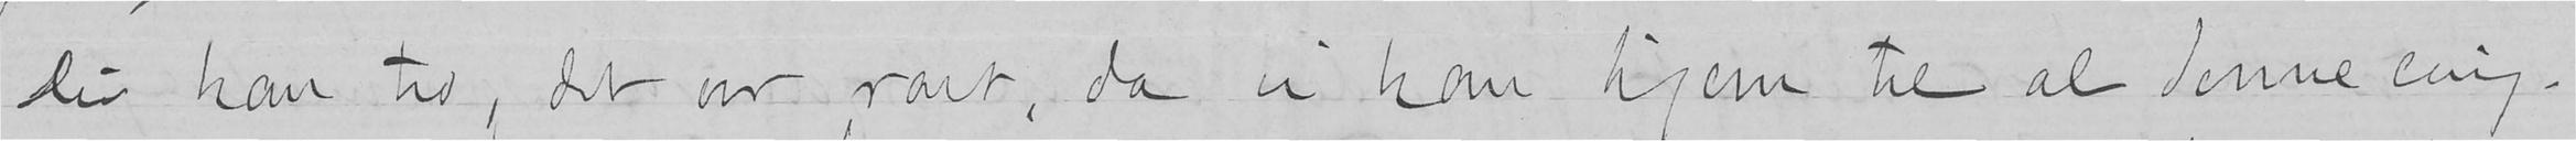Du kan tro, det var rart, da vi kom hjem til al denne enig-
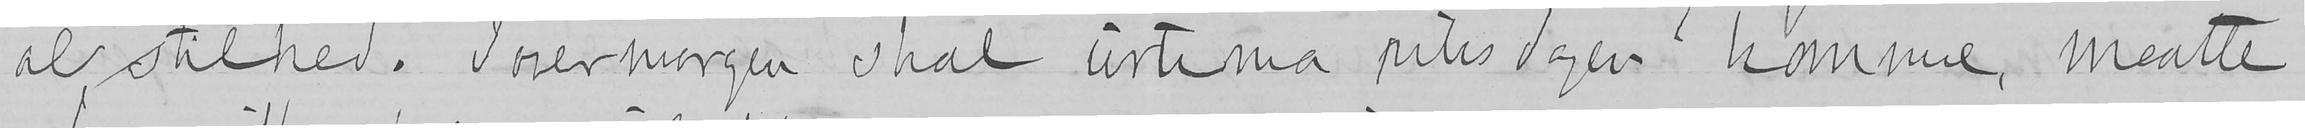al stilhed. Iovermorgen skal urtima riksdagen komme, maatte
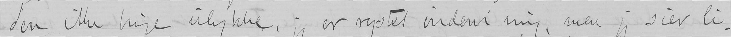den ikke bringe ulykke, jeg er rystet indeni mig, men jeg sier li-
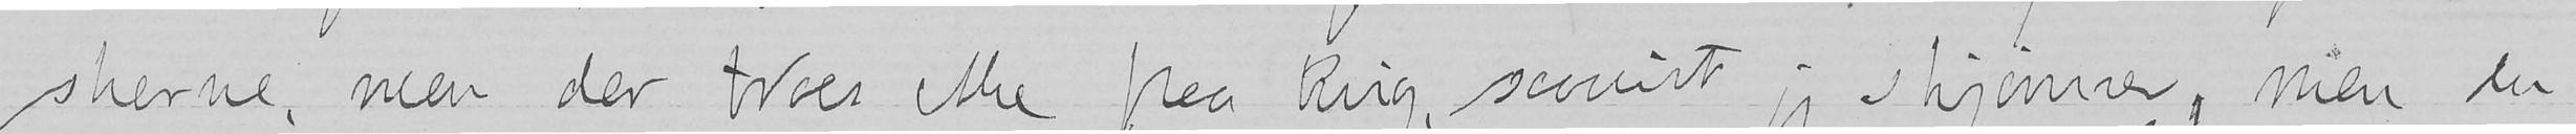skerne, men der troes ikke paa krig, saavidt jeg skjønner, men du
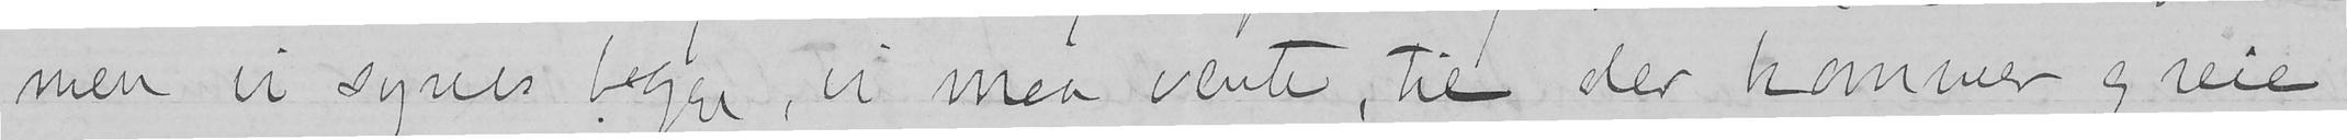men vi synes begge, vi maa vente, til der kommer greie
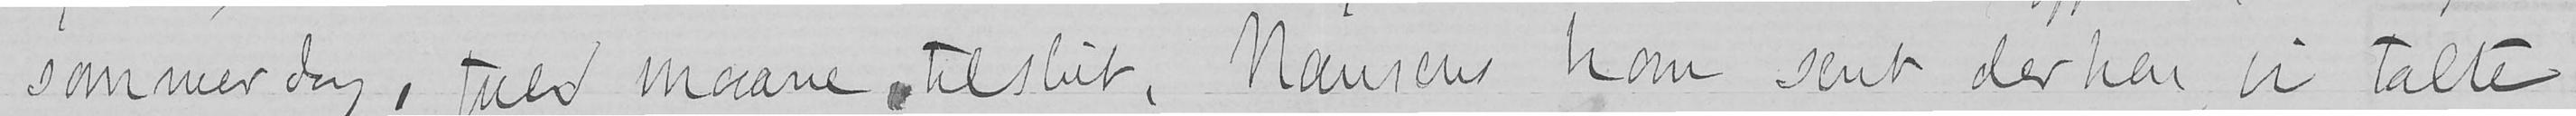sommerdag, fuld Maane tilslut, Nansens kom sent derhen, vi talte
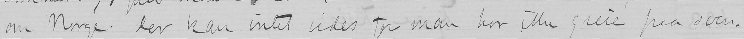om Norge. Der kan intet vides for man har ikke greie paa sven-
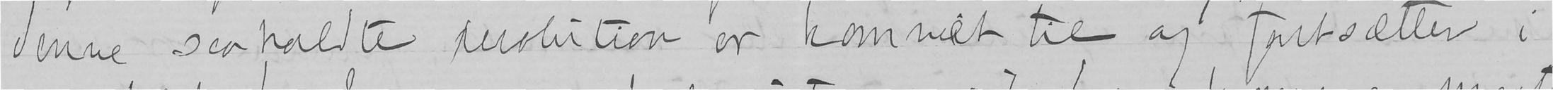denne saakaldte revolution er kommet til og fortsætter i
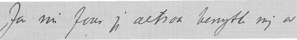Ja nu faar jeg altsaa benytte mig av
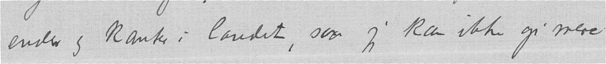ender og kanter i landet saa jeg kan ikke gi mere
